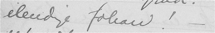elendige Johan! -
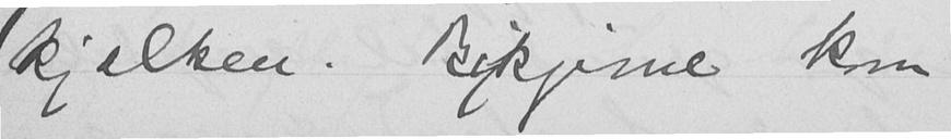kjelken. Bikjerne kom
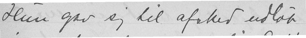Hun gav sig til afsked udløb
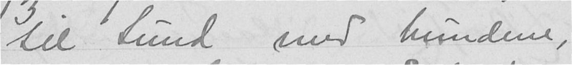til Sund med hundene,
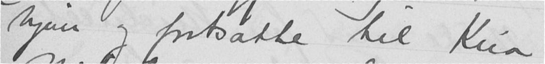hjem og fortsatte til Kria
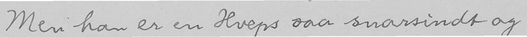Men han er en Hveps saa snarsindt og
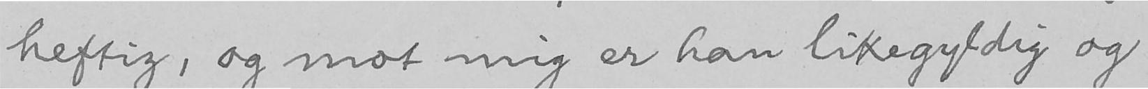heftig, og mot mig er han likegyldig og
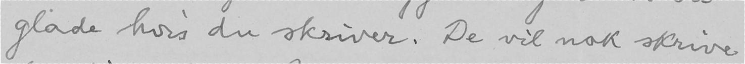glade hvis du skriver. De vil nok skrive
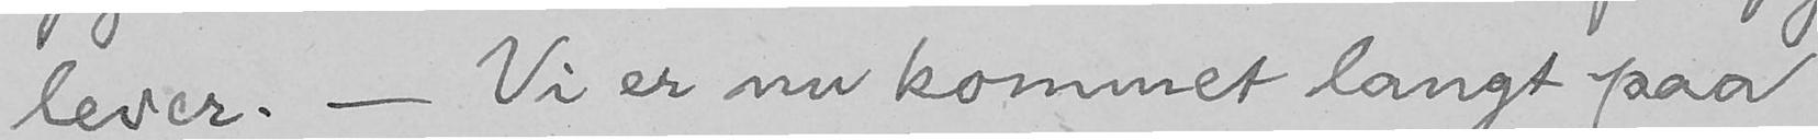lever. - Vi er nu kommet langt paa
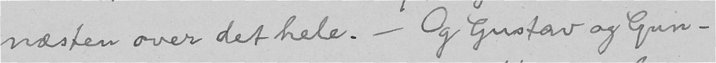næsten over det hele. - Og Gustav og Gun-
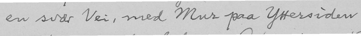en svær Vei, med Mur paa Yttersiden
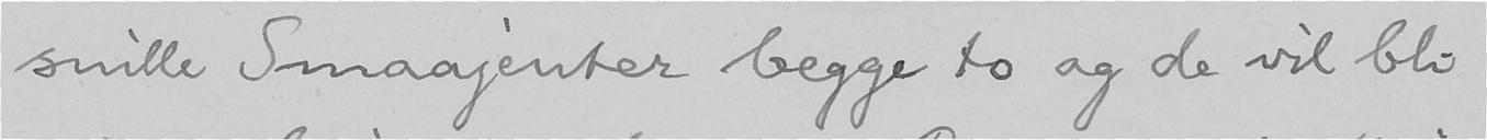snille Smaajenter begge to og de vil bli
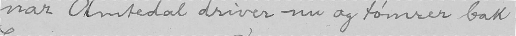nar Amtedal driver nu og tømrer bak
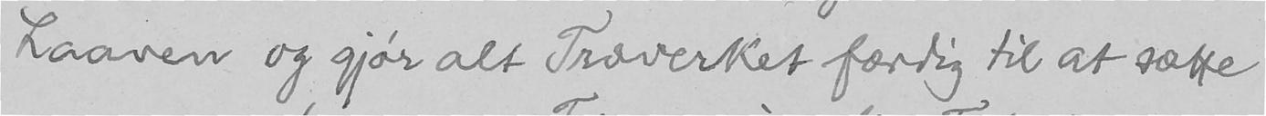Laaven og gjør alt Træverket færdig til at sætte
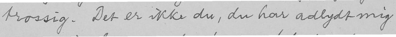trossig. Det er ikke du, du har adlydt mig
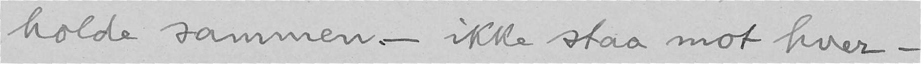holde sammen - ikke staa mot hver-
